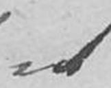et
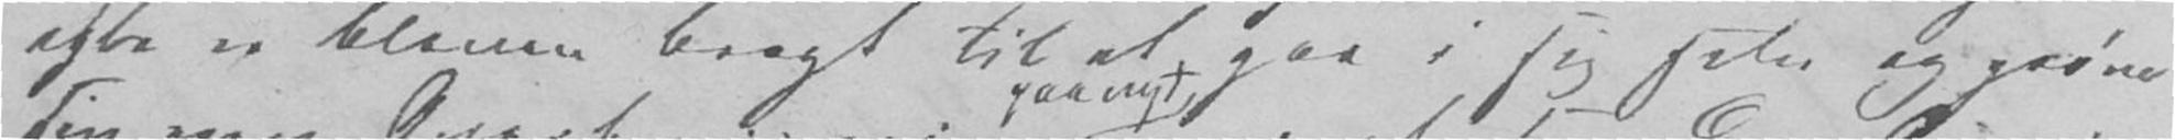ofte er bleven bragt til at gaa i sig selv og prøve
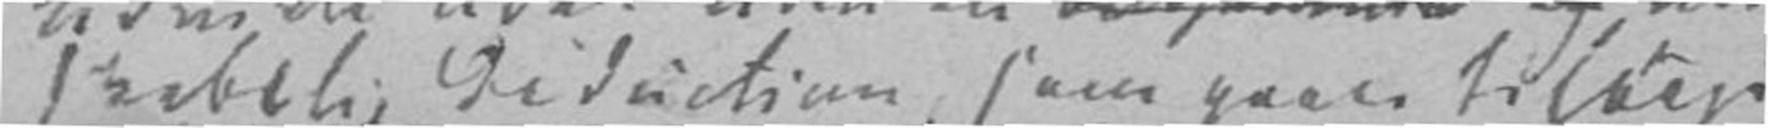skabelig Deduction, som gaaer tilbage
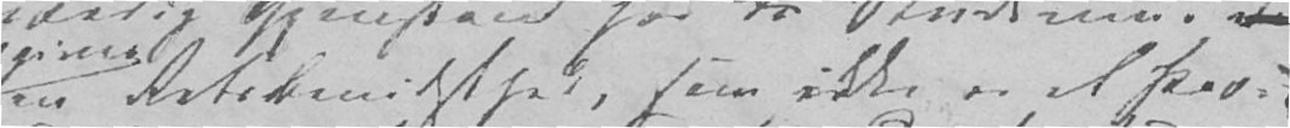en Retsbevidsthed, som ikke er et Pro-
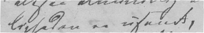ligheden er usandt,
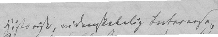Historisk, videnskabelig Interesse,
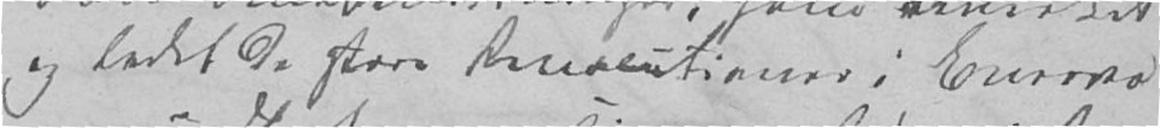og ledet de store Revolutioner i Europa
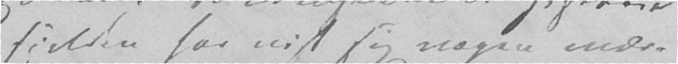sjelden har vist sig nogen mær-
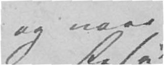og naar
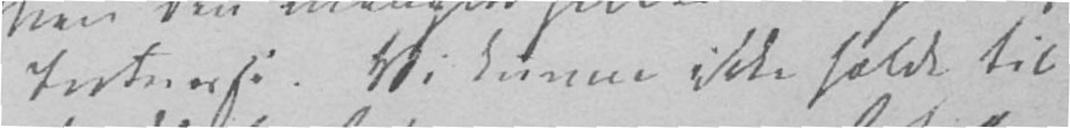Interesse. Vi kunne ikke holdt til-
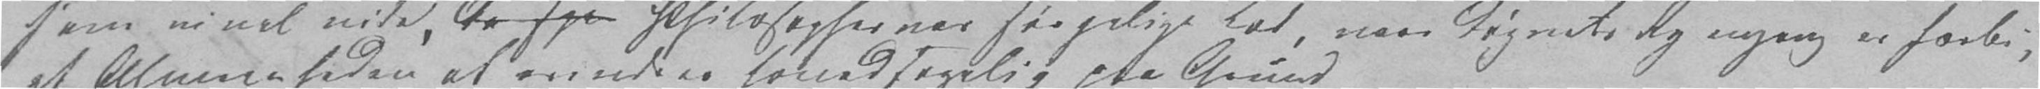som vi vel vide, Philosophernes sørgelige Lod, naar Døgnets Ry engang er forbi,
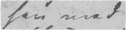han med
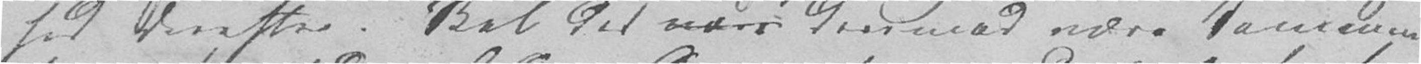hed derefter. Skal det derimod være Sammen-
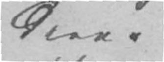deres
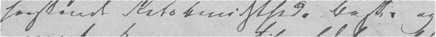herskende Retsbevidstheds Basis og
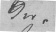der,
